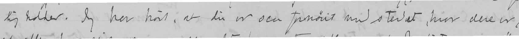lig holder. Jeg har hørt, at du er saa fornøiet med stedet, hvor dere er,
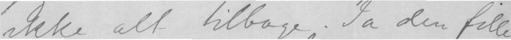ikke alt tilbage. Ja den fille
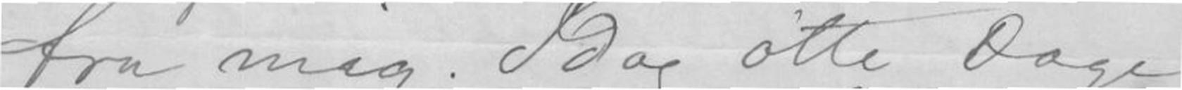fra mig. Idag otte Dage
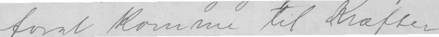forat komme til Kræfter
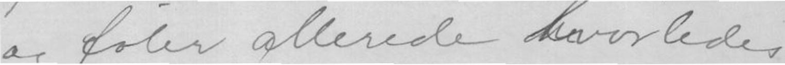og føler allerede hvorledes
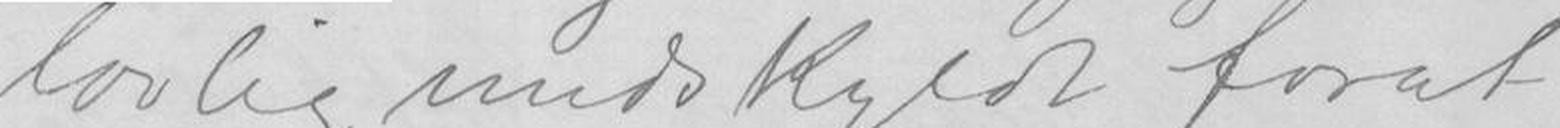lovlig undskyldt forat
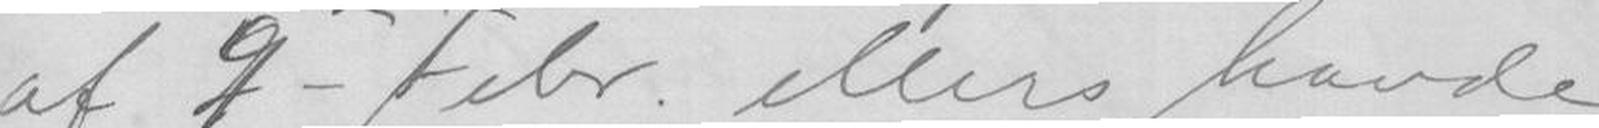af 9de Febr. ellers havde
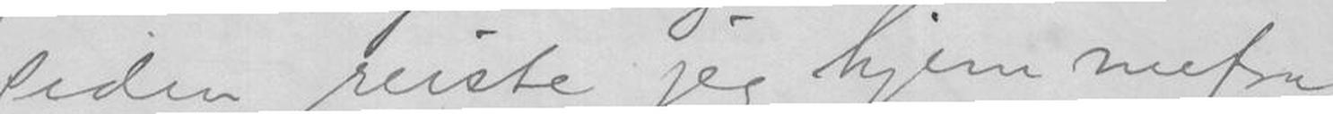siden reiste jeg hjemmefra
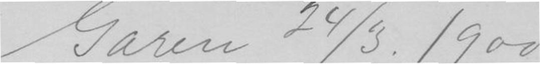Garen 24/3.1900
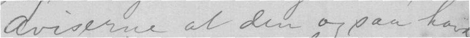Aviserne at den ogsaa havde
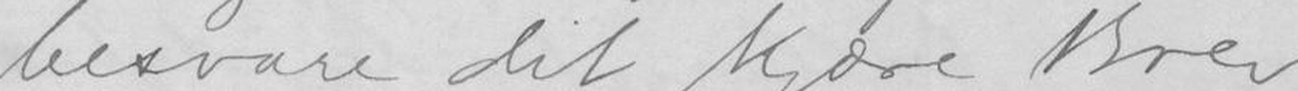besvare dit kjære Brev
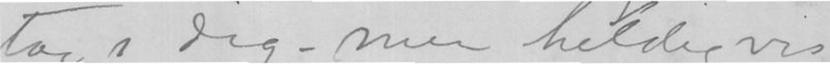tag i dig - men heldigvis
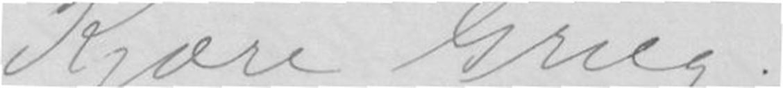Kjære Grieg!
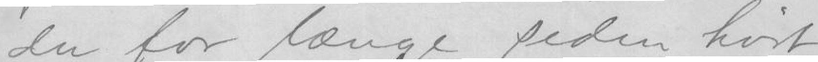du for længe siden hørt
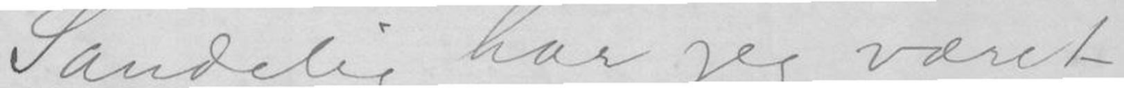Sandelig har jeg været
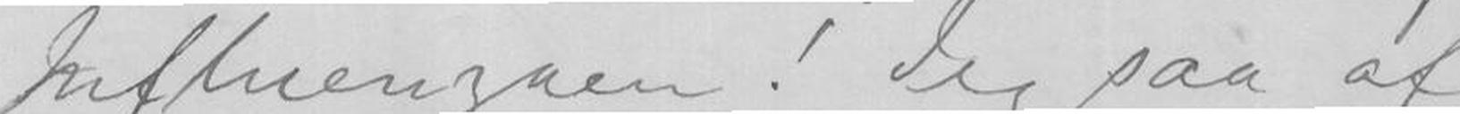Influenzaen! Jeg saa af
